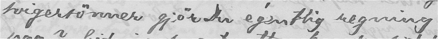svigersønner gjør Du egentlig regning
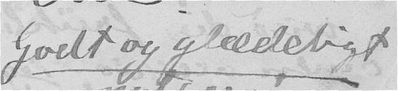Godt og glædeligt
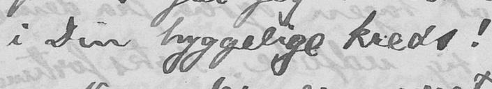i Din hyggelige kreds!
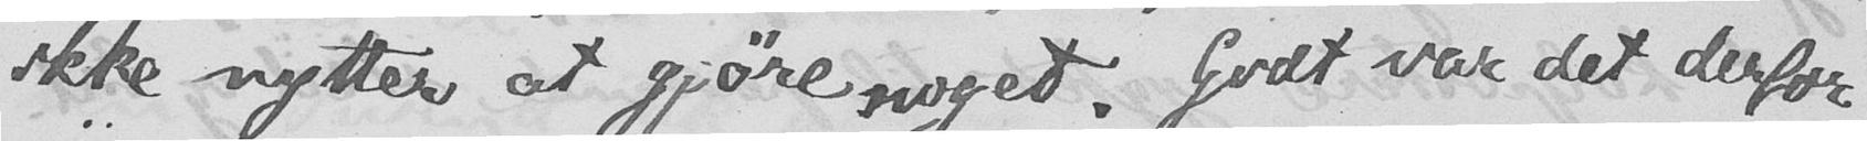ikke nytter at gjøre noget. Godt var det derfor
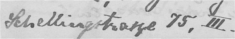Schellingstrasse 75, III.
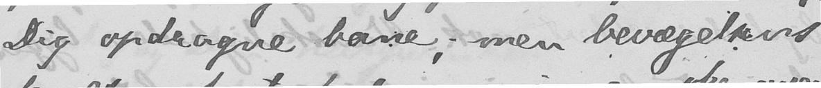Dig opdragne bane; men bevægelsens
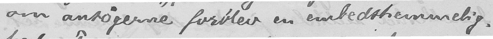om ansøgerne forblev en embedshemmelig-
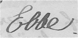Ebbe.
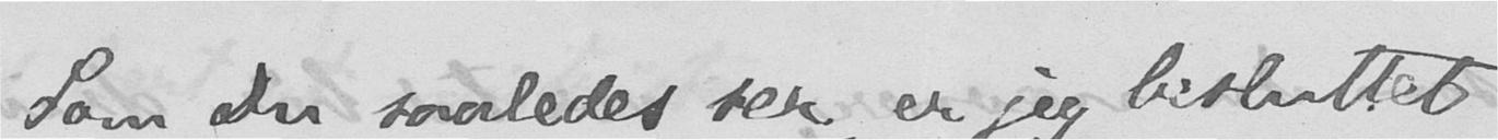Som Du saaledes ser, er jeg besluttet
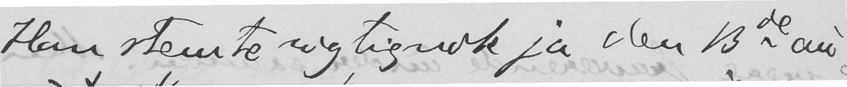Han stemte rigtignok ja den 13de au-
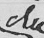da
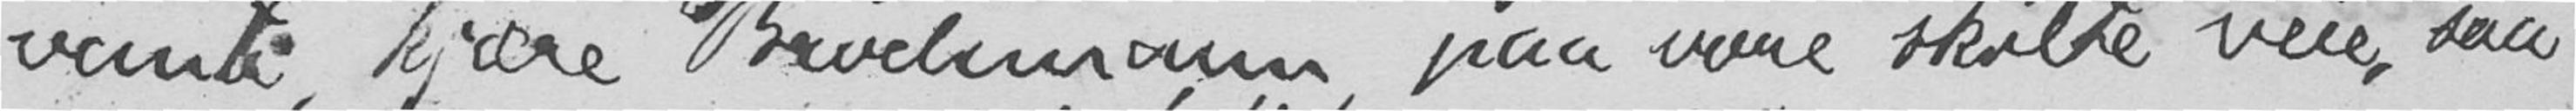vanti, kjære Brochmann, paa vore skilte veie, saa
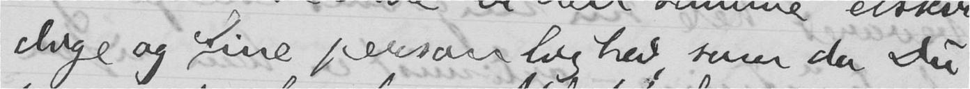dige og fine personlighed, som da Du
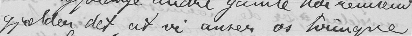gjælder det, at vi anser os tvungne
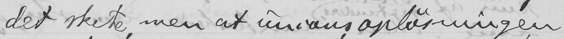det skete, men at unionsopløsningen
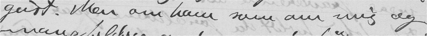gust. Men om ham som om mig og
